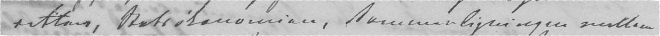retten, Statsøkonomien, Sammenligningen mellem
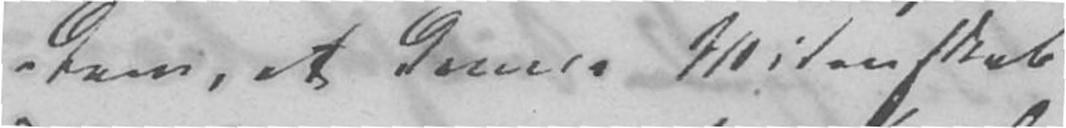Dere, at denne Videnskab
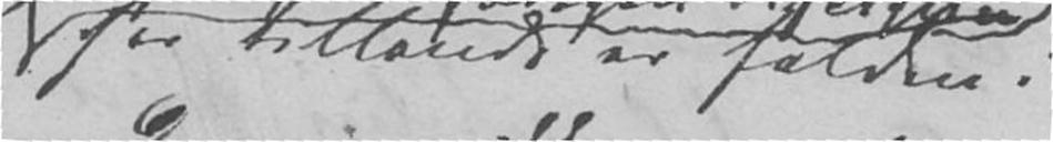her tillands er falden i
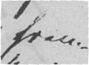frem-
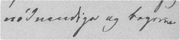nødvendige og begrun-
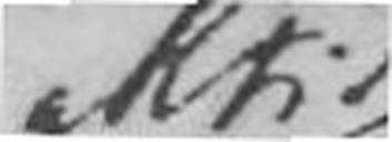altid
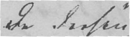De derhen
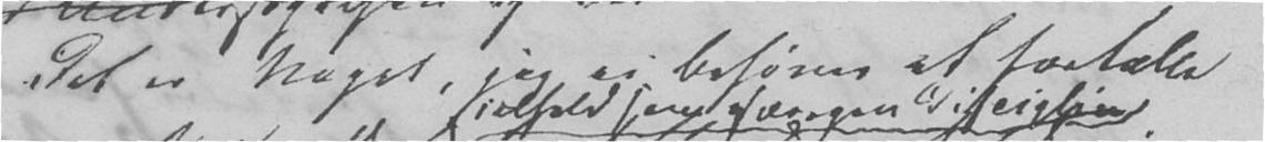Det er Noget, jeg ei behøver at fortælle
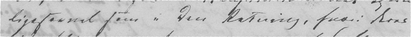ligesaavel som i den Retning, hvori deres
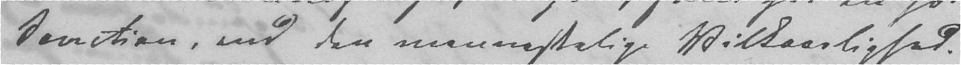Sanction, end den menneskelige Vilkaarlighed.
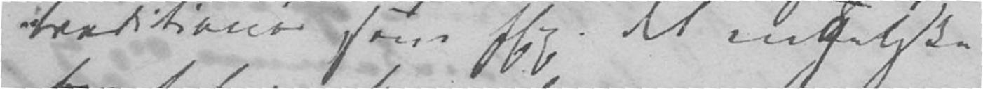Traditioner som fEx. det engelske
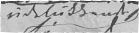udelukkende
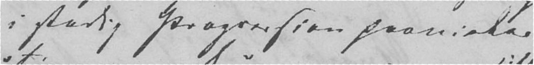i stadig Progression paavirker
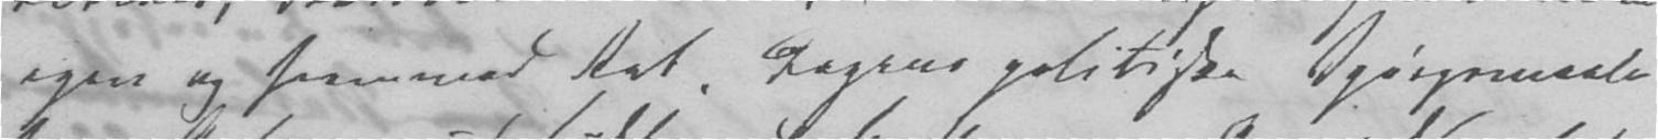egen og fremmed Ret, Dagens politiske Spørgsmaal
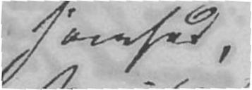somhed,
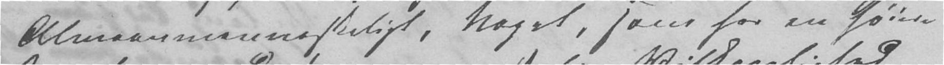Almeenmenneskeligt, Noget, som har en høiere
